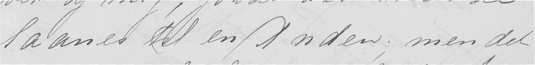laanes til en Anden; men det
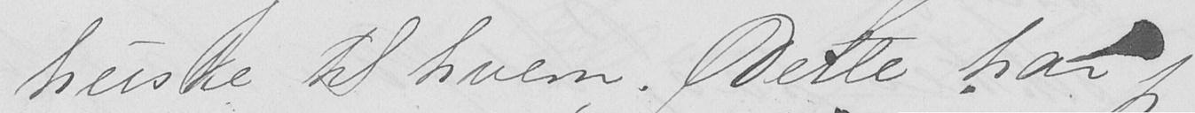huske til hvem. Dette har jeg
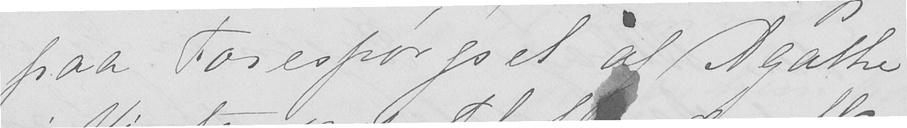paa Forespørgsel af Agathe
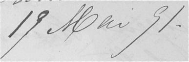19 Mai 91.
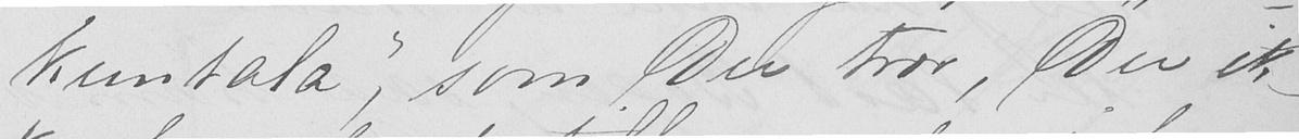kuntala", som Du tror, Du ik-
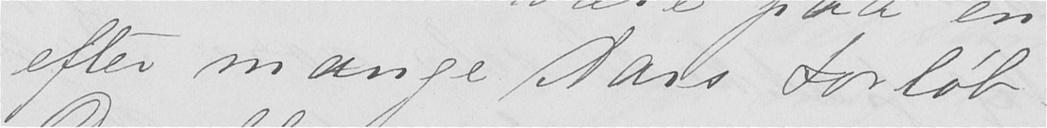efter mange Aars Forløb.
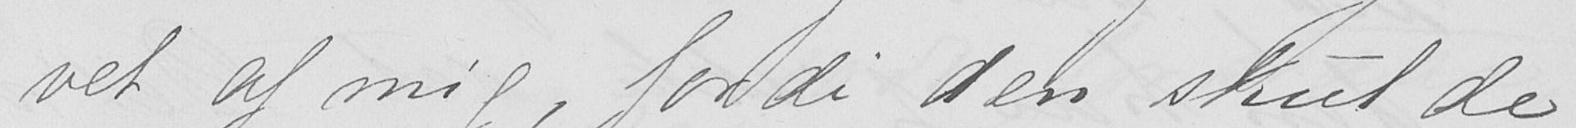vet af mig, fordi den skulde
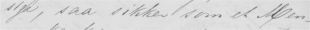sige, saa sikker som et Men-
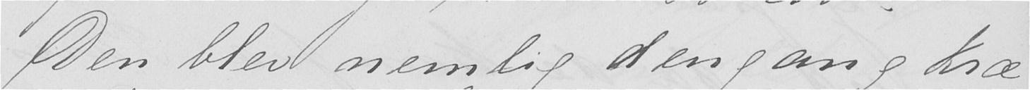Den blev nemlig dengang kræ-
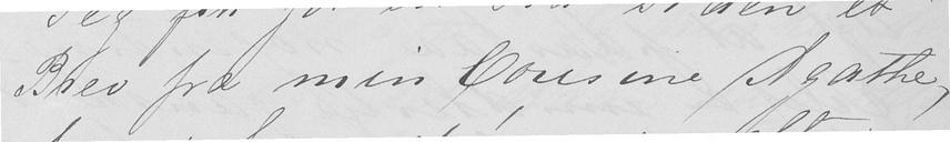Brev fra min Cousine Agathe,
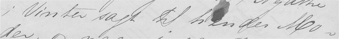i Vinter sagt til hendes Mo-
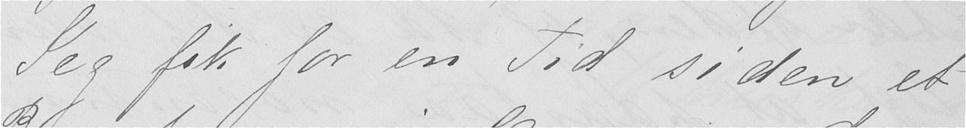Jeg fik for en Tid siden et
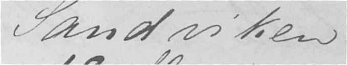Sandviken
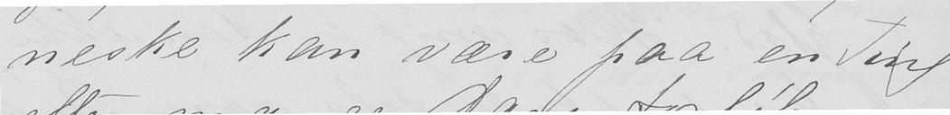neske kan være paa en Ting
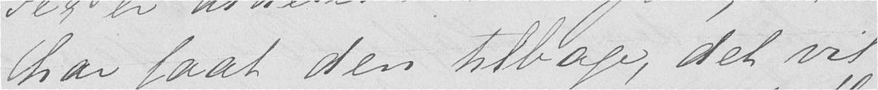har faat den tilbage, det vil
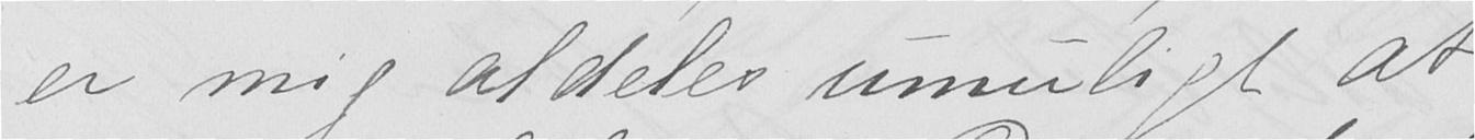er mig aldeles umuligt at
